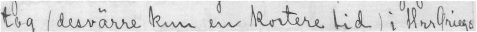tog (desvärre kun en kortere tid) i Hrr Griegs
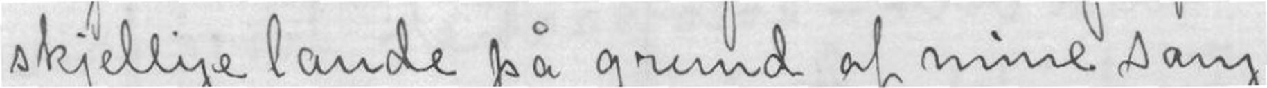skjellige lande på grund af mine sang-
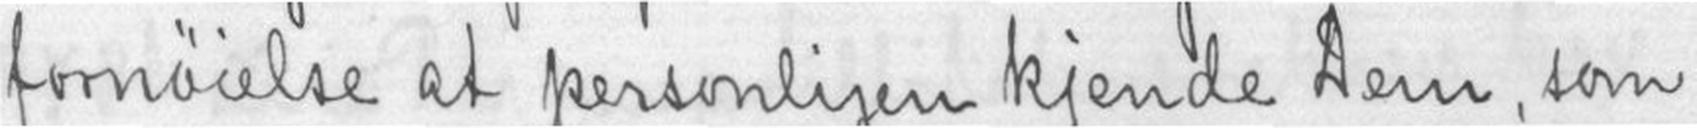fornöielse at personligen kjende Dem, som
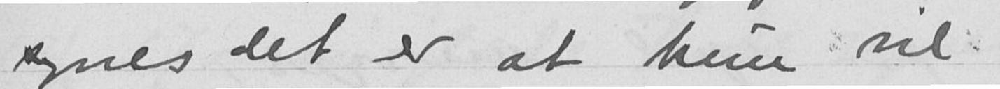synes det er at hun vil
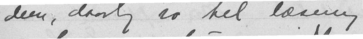dem, daarlig ro til læsing
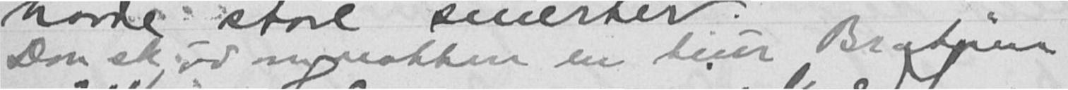Don skjød om natten en tiur Bratøen
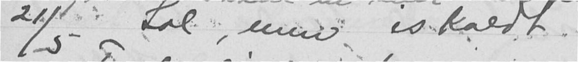21/5 Sol, men iskoldt.
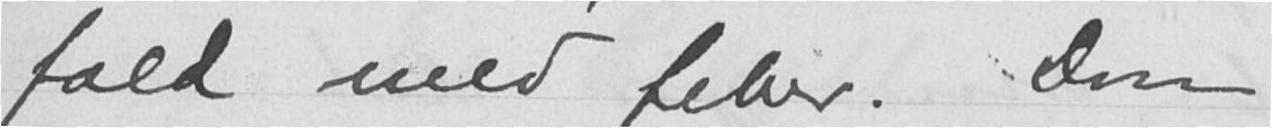fald med feber. Don
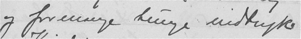og formange tunge indtryk.
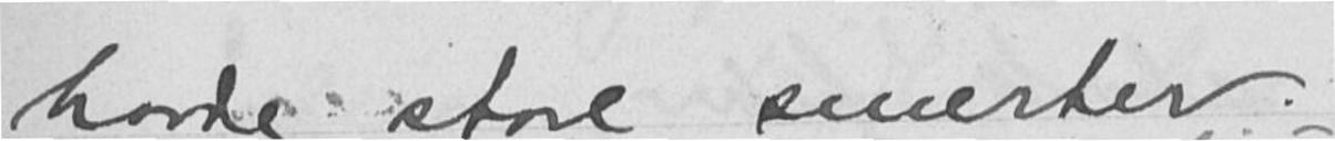havde store smerter.
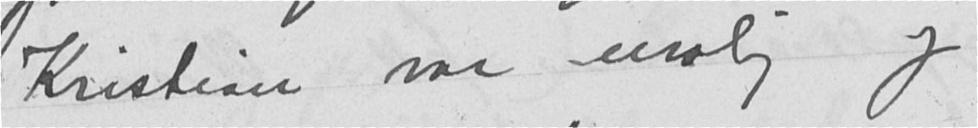Kristian var urolig og
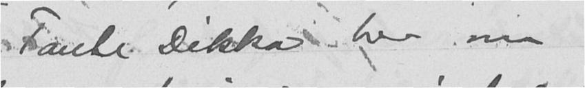Tante Dikka her om
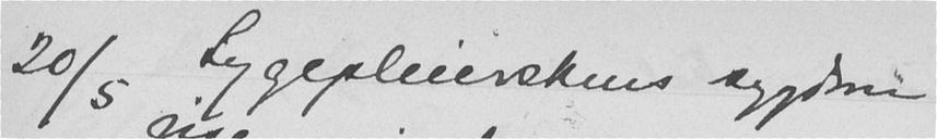20/5 Sygepleierskens sygdom
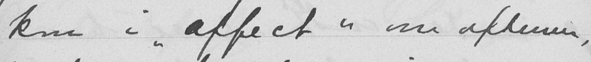kom i "affect" om aftenen,
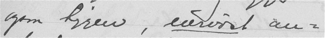ogsaa Siggen, nervøst an-
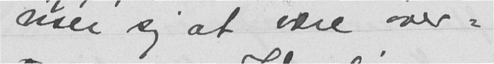viser sig at være over-
